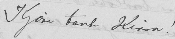Kjære tante Kirsa!
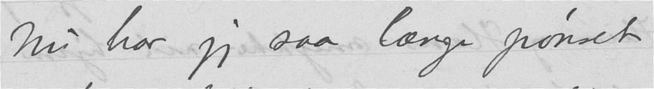Nu har jeg saa længe pønsket
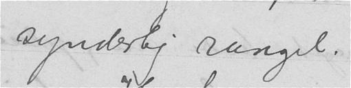synderlig rangel.
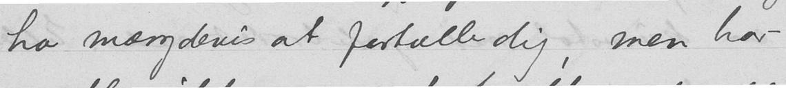ha mængdevis at fortælle dig, men har
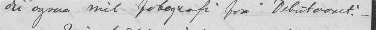du ogsaa mit fotografi fra "Debutaaret". –
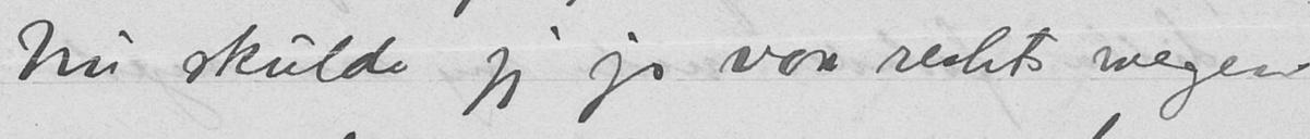Nu skulde jeg jo von rechts wegen
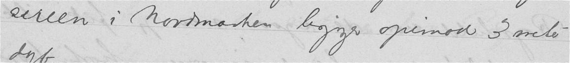sneen i Nordmarken ligger opimod 3 meter
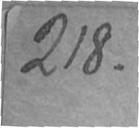218.
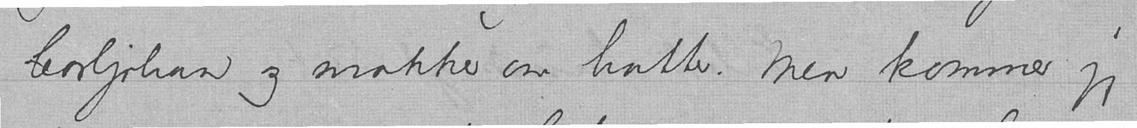Carljohan og snakker om hatter. Men kommer jeg
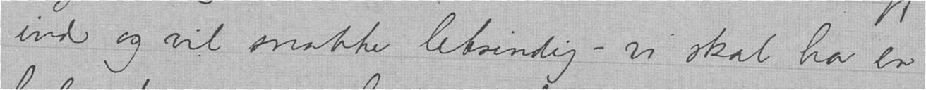ind og vil snakke letsindig - vi skal ha en
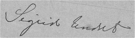Sigrid Undset
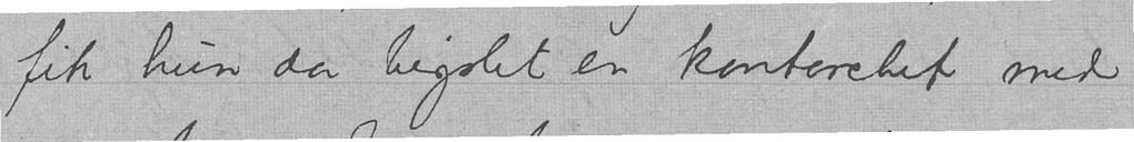fik hun da bigslet en kontorchef med
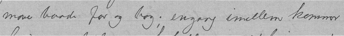mave baade for og bag; engang imellem kommer
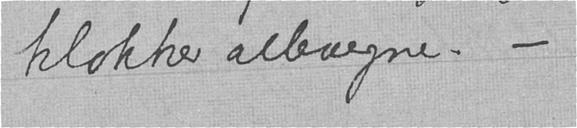klokker allevegne. -
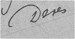Deres
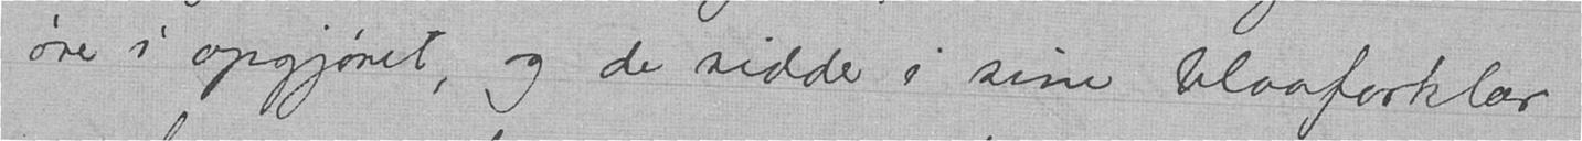øre i opgjøret, og de sidder i sine blaaforklær
